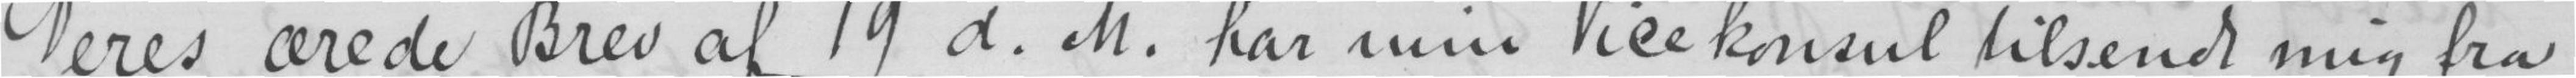Deres ærede Brev af 19 d.M. har min Vicekonsul tilsendt mig fra
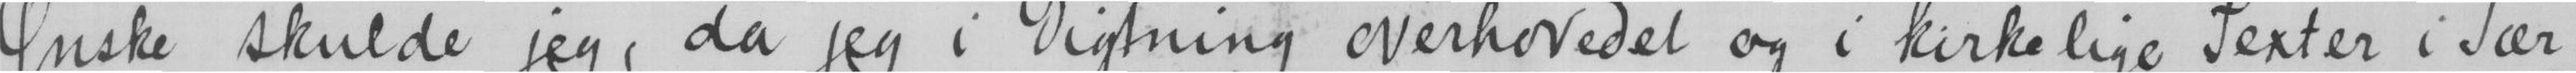Ønske skulde jeg, da jeg i Digtning overhovedet og i kirkelige Texter i Sær
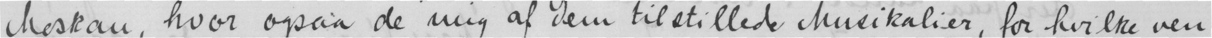Moskau, hvor ogsaa de mig af Dem tilstillede Musikalier, for hvilke ven-
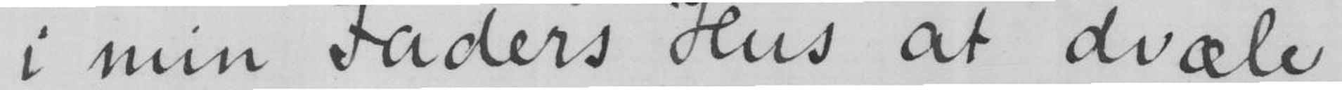i min Faders Hus at dvæle
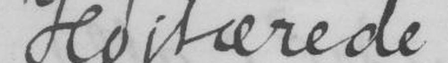Højtærede Consulat de Danemark
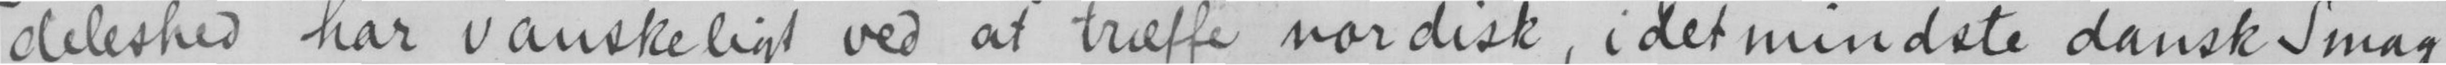deleshed har vanskeligt ved at træffe nordisk, i det mindste dansk Smag,
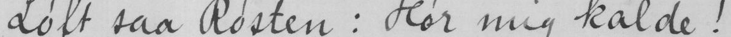Løft saa Røsten: Hør mig kalde!
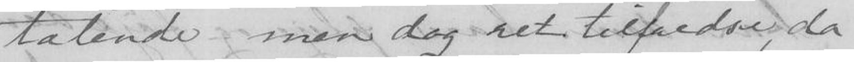talende men dog ret tilfredse, da
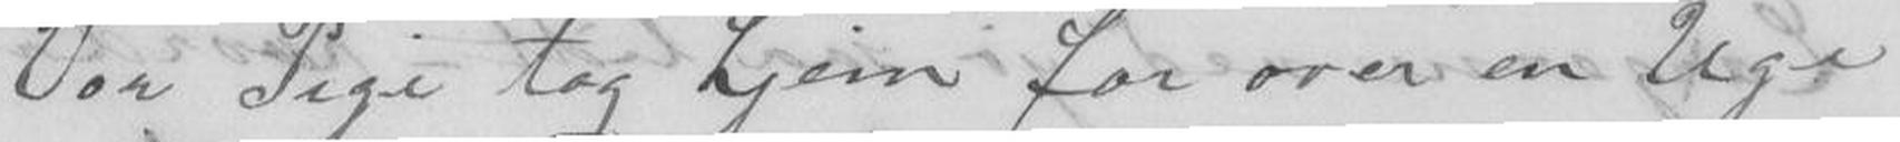Vor Pige tog hjem for oven en Uge
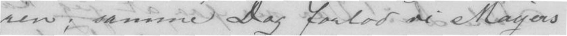ren; samme Dag forlod vi Mayers
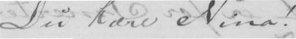Du kære Nina!
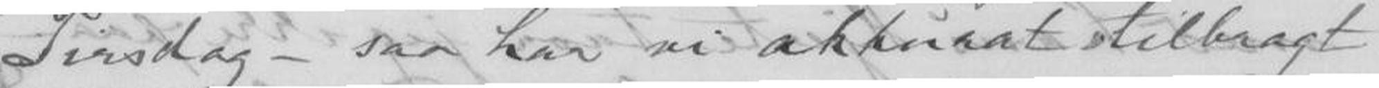Tirsdag - saa har vi akkurat tilbragt
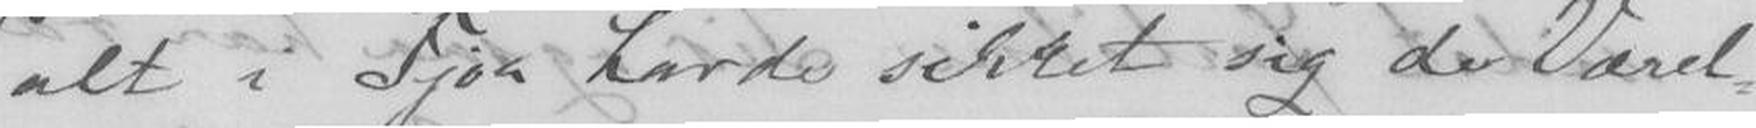alt i Fjor havde sikret sig de Værel-
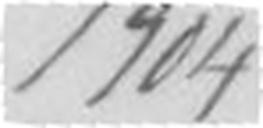1904
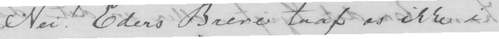Nei! Eders Breve traf os ikke i
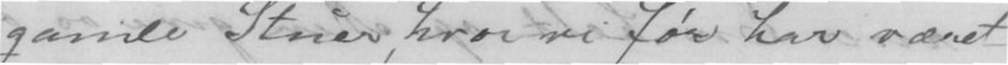gamle Stuer, hvor vi før har været
